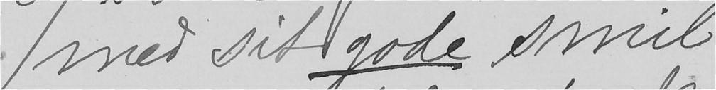med sit gode smil
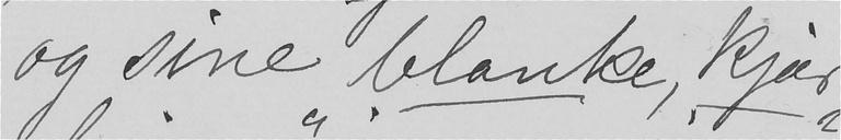og sine blanke, kjær-
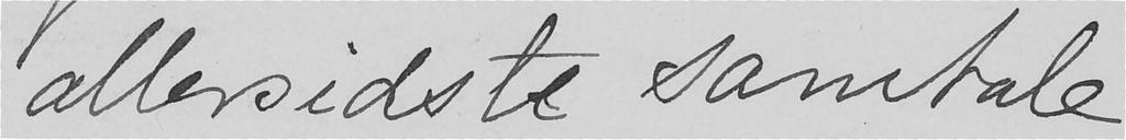allersidste samtale
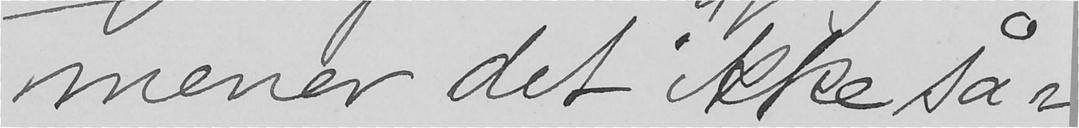mener det ikke så-
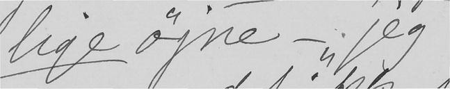lige øjne - "jeg
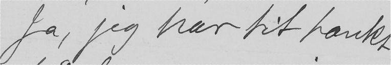Ja, jeg har tit tænkt
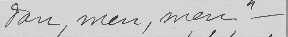dan, men, men" -
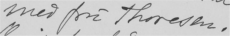med fru Thoresen.
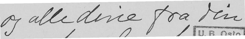og alle dine fra din
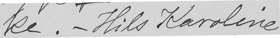ke. - Hils Karoline
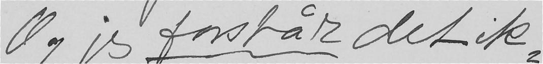Og jeg forstår det ik-
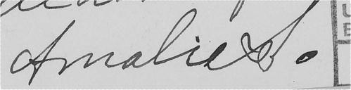Amalie S.
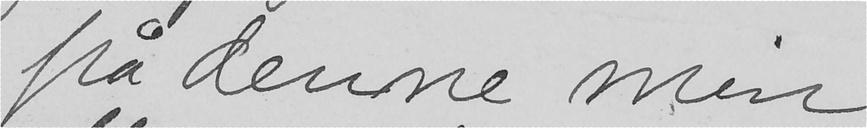på denne min
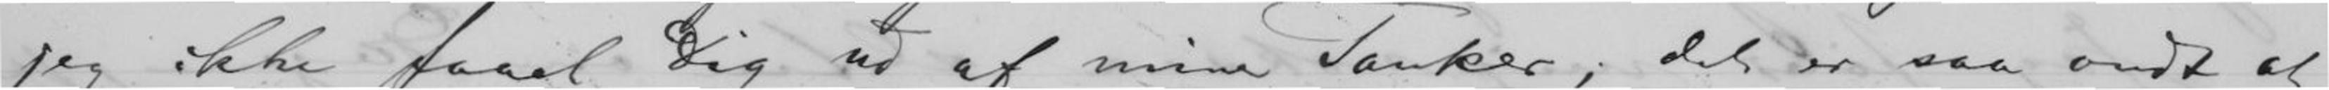jeg ikke faaet Dig ud af mine Tanker; det er saa ondt at
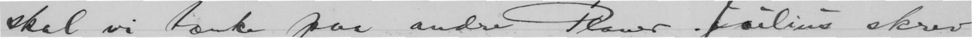skal vi tænke paa andre Planer. Julius skrev
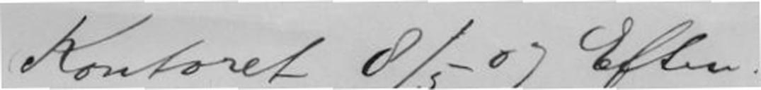Kontoret 8/5 07 Efterm.
